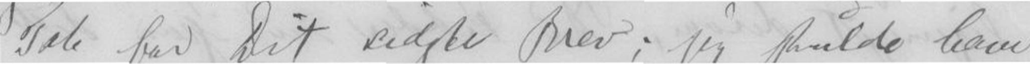Tak for Dit sidste Brev; jeg skulde have
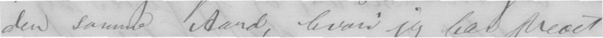den samme Aand, hvori jeg har skrevet
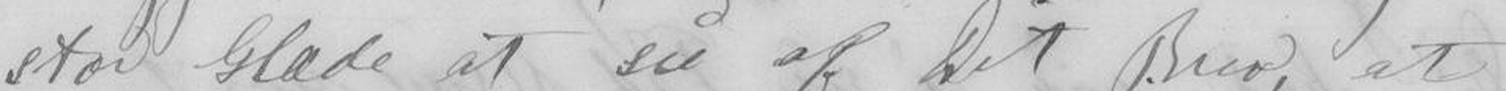stor Glæde at see af Dit Brev, at
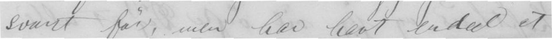svaret før, men har havt endeel at
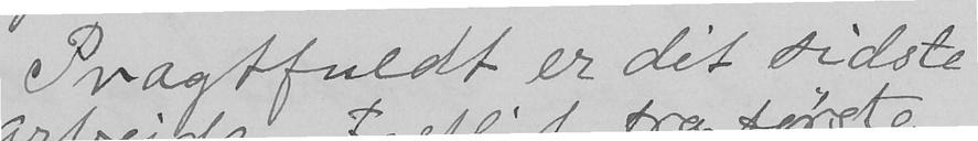Pragtfuldt er dit sidste
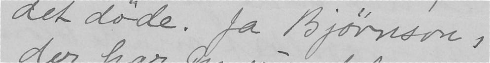det døde. Ja Bjørnson,
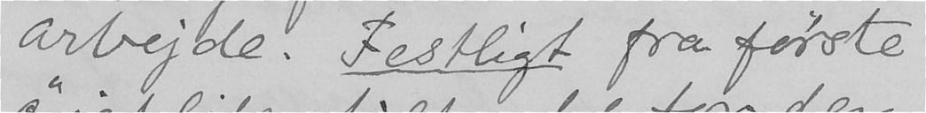arbejde. Festligt fra første
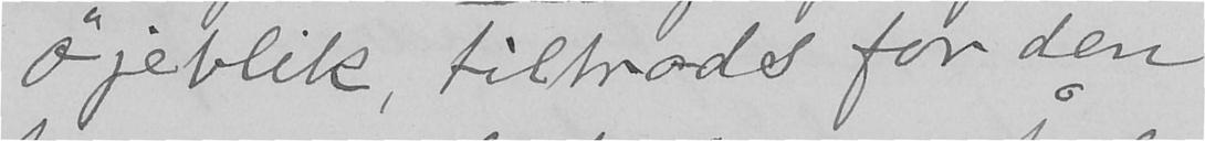øjeblik, tiltrods for den
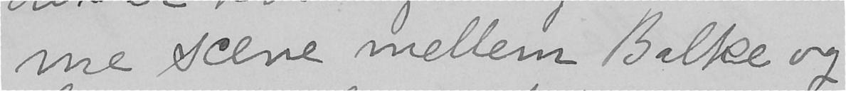me scene mellem Balke og
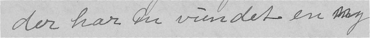der har Du vundet en ny
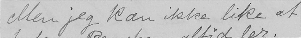Men jeg kan ikke like at
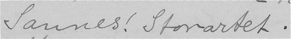Sannes! Storartet.
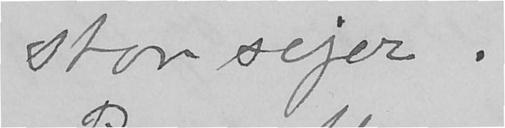stor sejer.
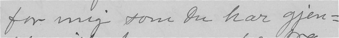for mig som Du har gjen-
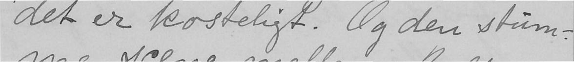det er kosteligt. Og den stum-
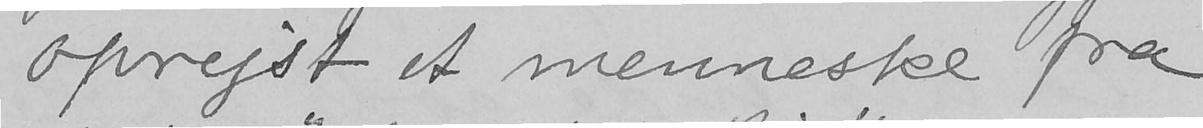oprejst et menneske fra
